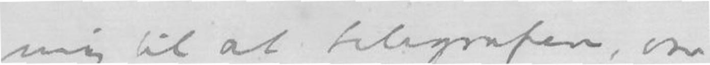mig til at telegrafere, var
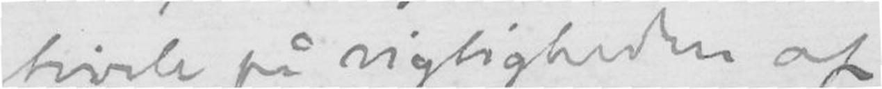tvivle på vigtigheden af
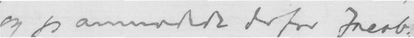og jeg annmodede derfor Jacob-
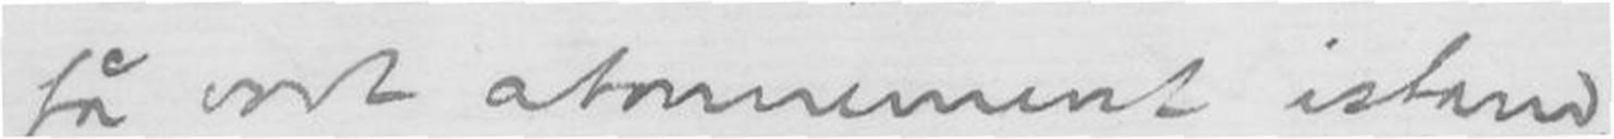få vort abonnement istand,
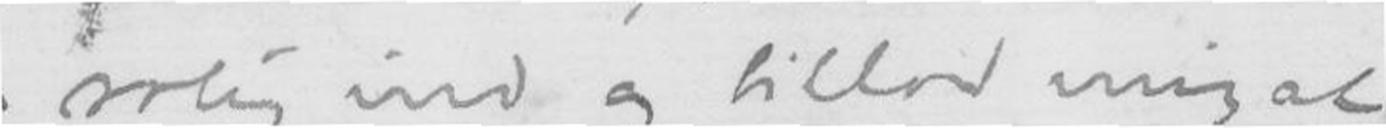rolig ind og tillod mig at
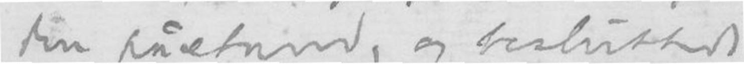den påstand, og besluttede
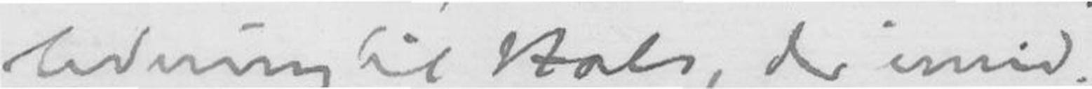ledning til Hals, der imid-
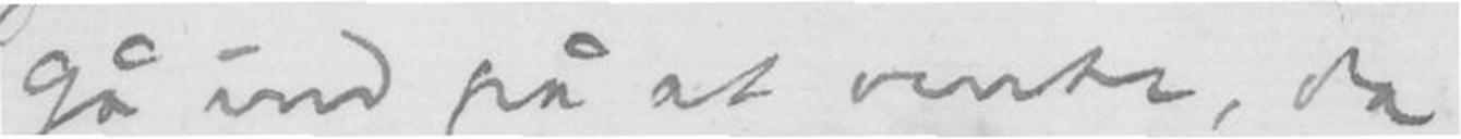gå ind på at vente, da
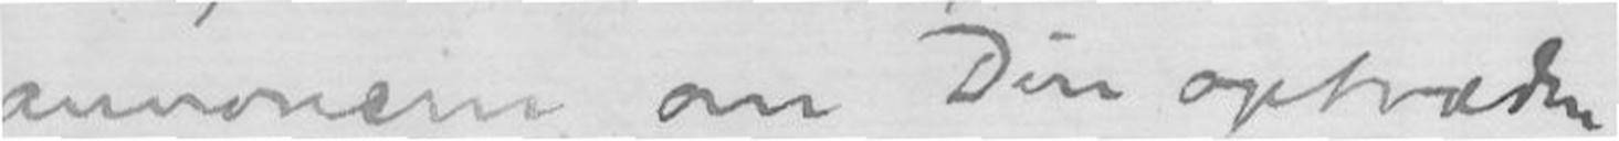annoncen om Din optræden.
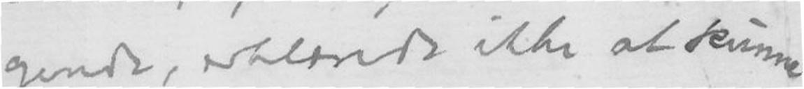gende, erklærede ikke at kunne
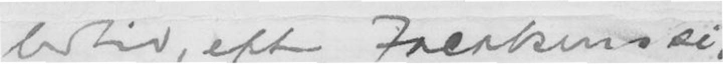lertid, efter Jacobsen si-
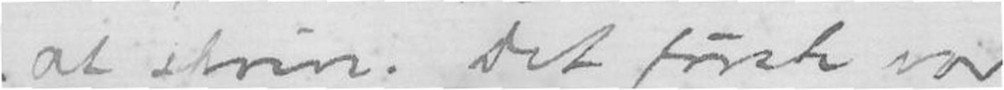at skrive. Det første var
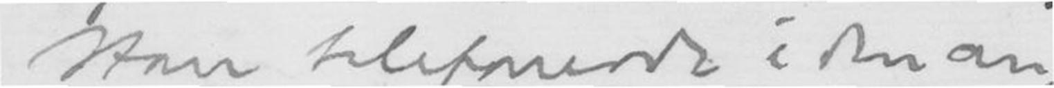Han telefonerede i den an-
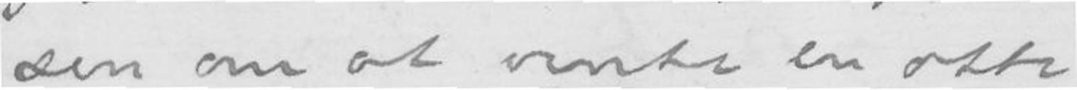sen om at vente en otte
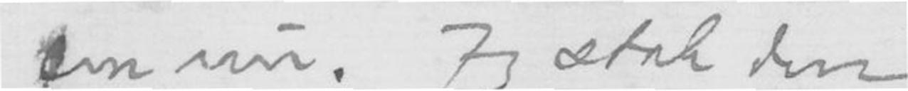cen nu. Jeg stak den
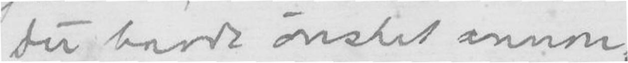Du havde ønsket annon-
